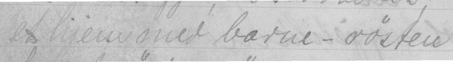et hjem med barne-røsten
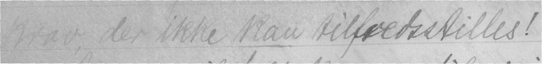krav, der ikke kan tilfredsstilles!
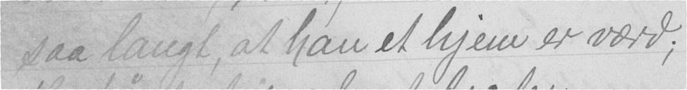saa langt, at han et hjem er værd;
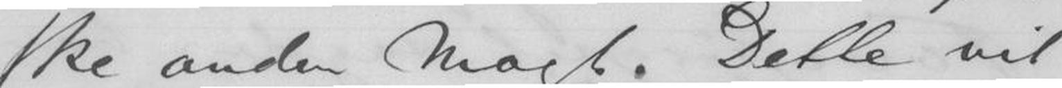ske anden Magt. Dette vil
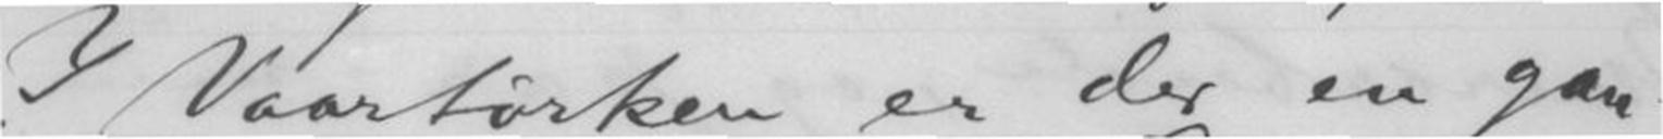I Vaartørken er der en gan-
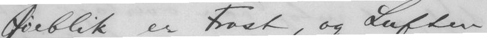Øieblik er Frost, og Luften
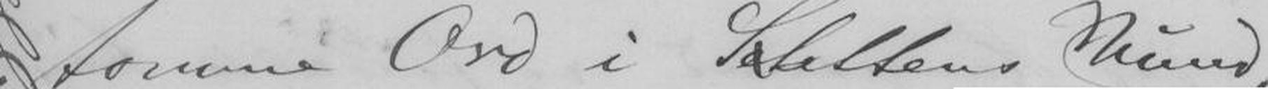tomme Ord i Slettens Mund,
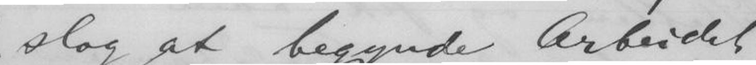slog at begynde Arbeidet
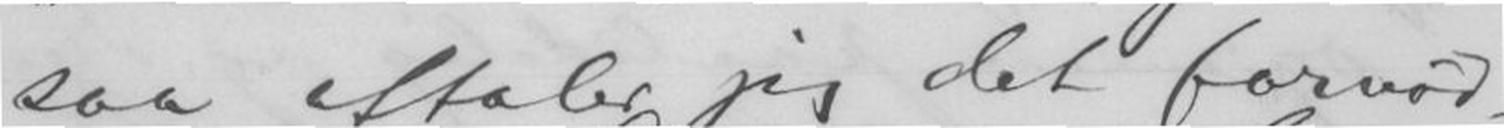saa aftaler jeg det fornød-
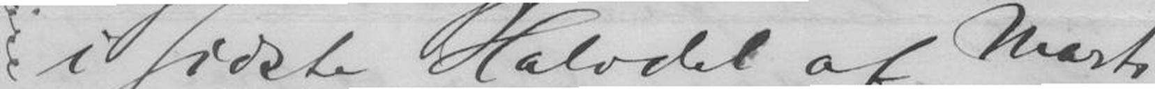i sidste Halvdel af Marts
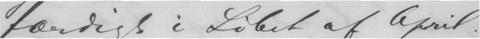færdigt i Løbet af April.
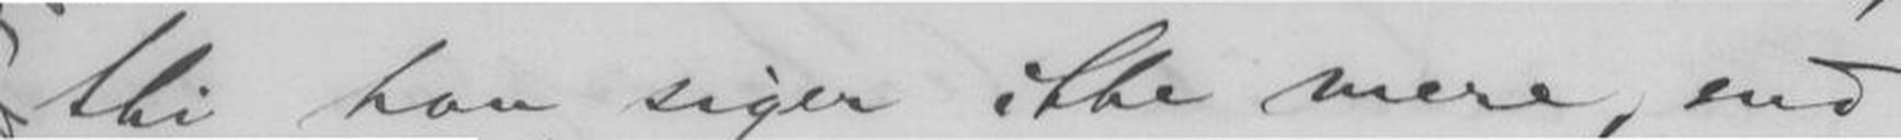thi han siger ikke mere, end
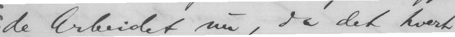de Arbeidet nu, da det hvert
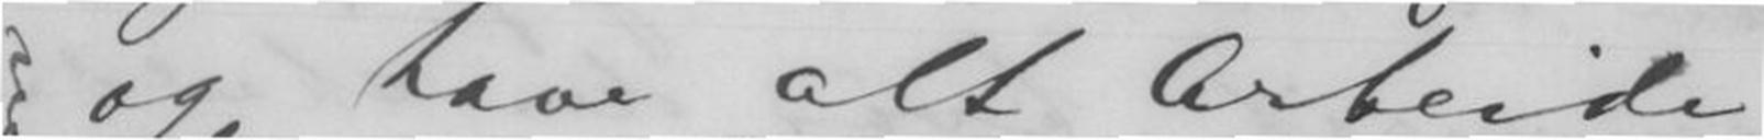og have alt Arbeide
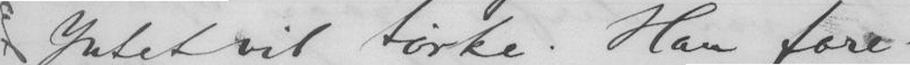Intet vil tørke. Han fore-
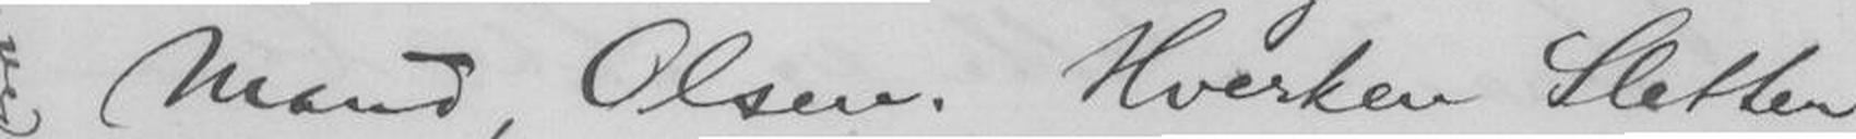Mand, Olsen. Hverken Sletten
